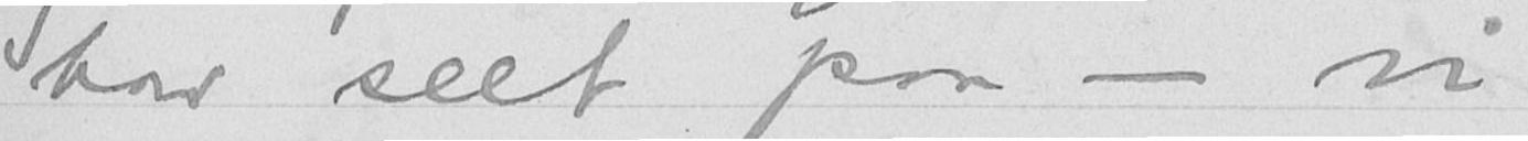har seet paa - vi
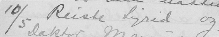10/5 Reiste Sigrid og
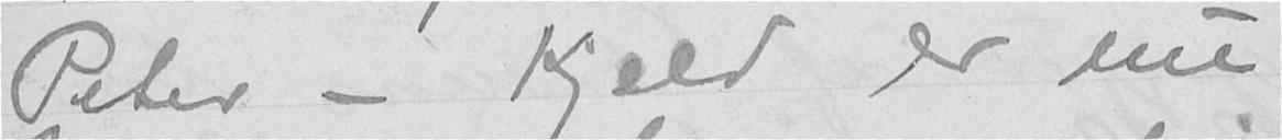Peter - Kjeld er nu
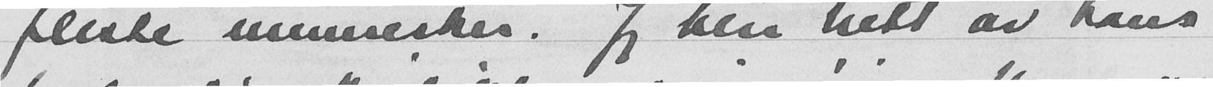fleste mennesker. Jeg blir trett av hans
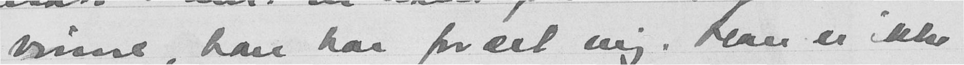rinne, han har foræret mig. Han er ikke
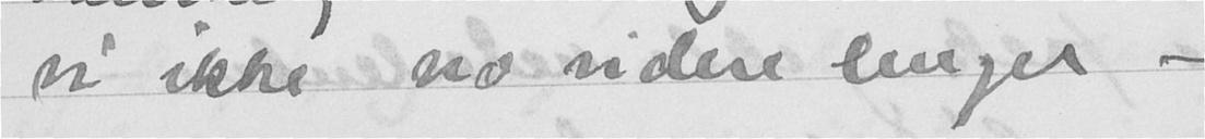vi ikke no videre lenger -
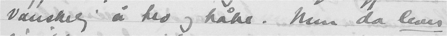vanskelig å tro og håpe. Men da lever
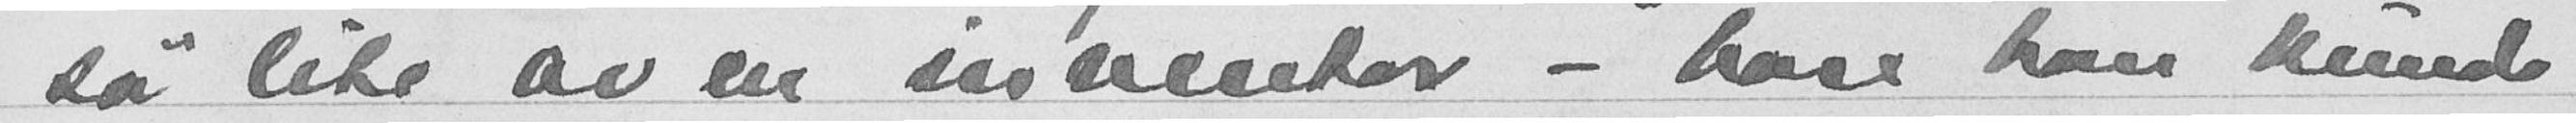så lite av en investor - bare han kunde
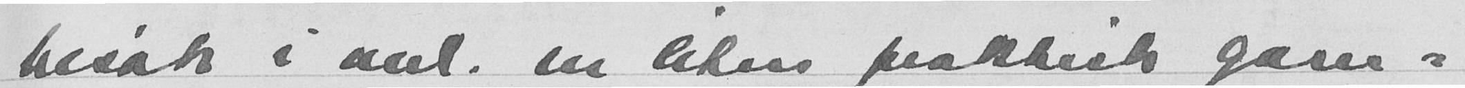besøk i anl. en liten praktisk gave-
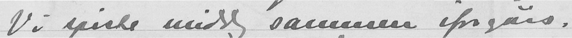Vi spiste middag sammen iforgårs.
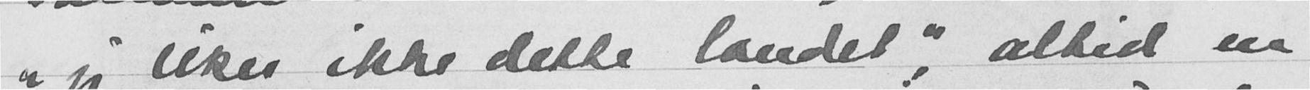"jeg liker ikke dette landet", altid en
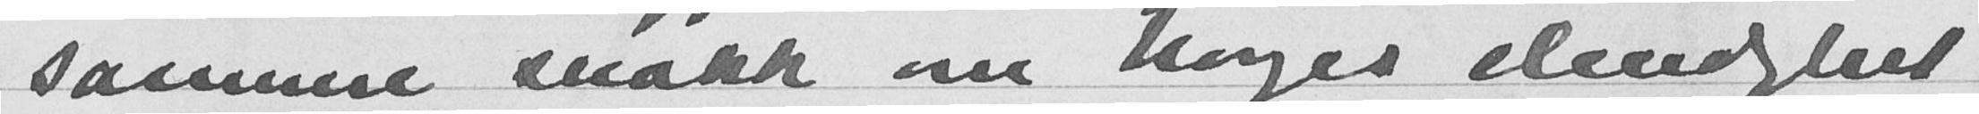samme snakk om Norges elendighet
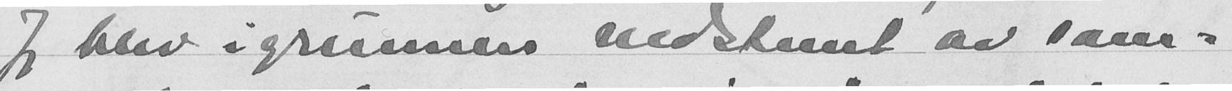Jeg blev i grunnen nedstemt av sam-
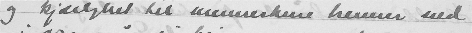og kjærlighet til menneskene brenner ned
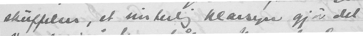skuffelser, et virkelig klarsyn gjør det
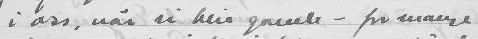i oss, når vi blir gamle - for mange
